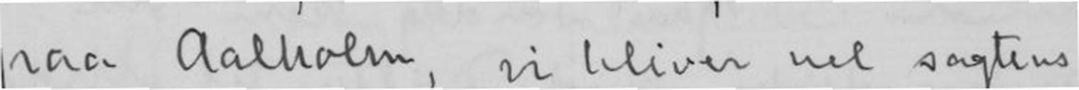paa Aalholm, vi bliver vel sagtens
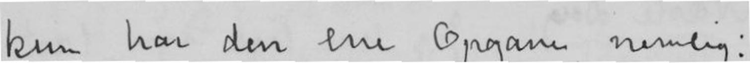kun har den ene Opgave, nemlig:
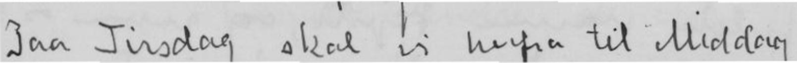Saa Tirsdag skal vi herfra til Middag
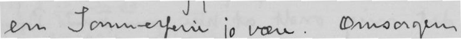en Sommerferie jo være. Omsorgen
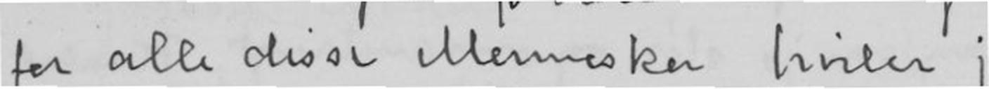for alle disse Mennesker hviler jo
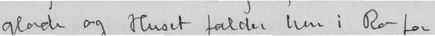glade og Huset falder hen i Ro for
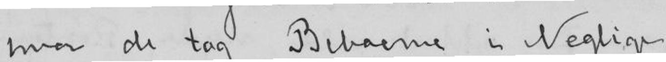hvor de tog Beboerne i Neglige,
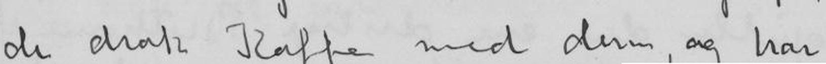de drak Kaffe med dem, og har
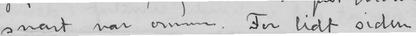snart var omme. For lidt siden
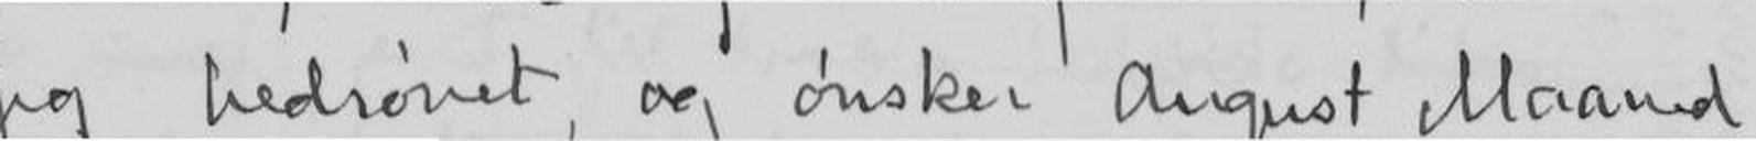jeg bedrøvet, og ønsker August Maaned
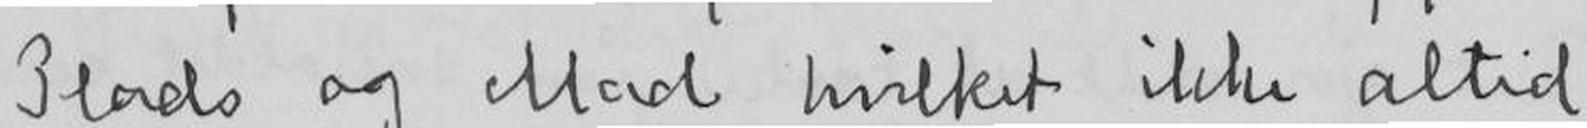Plads og Mad hvilket ikke altid
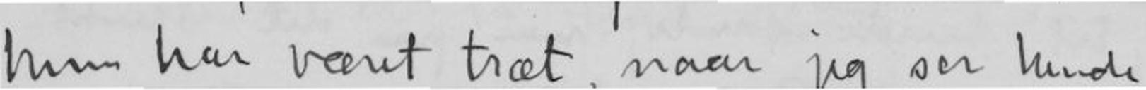hun har været træt, naar jeg ser hende
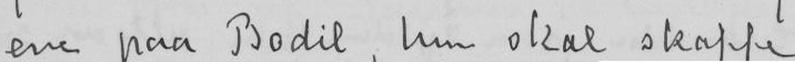ene paa Bodil, hun skal skaffe
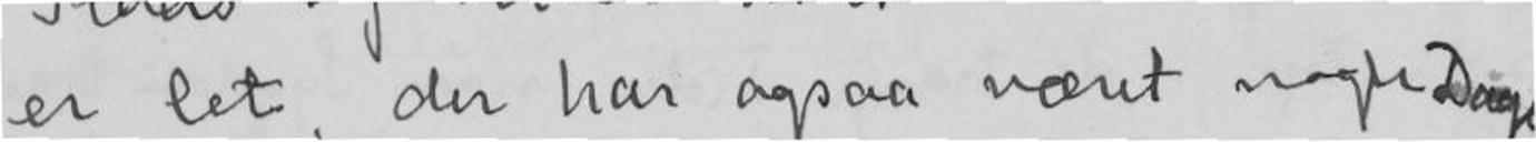er let, der har ogsaa været nogle Dage
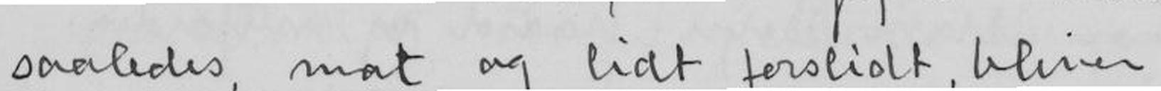saaledes, mat og lidt forslidt, bliver
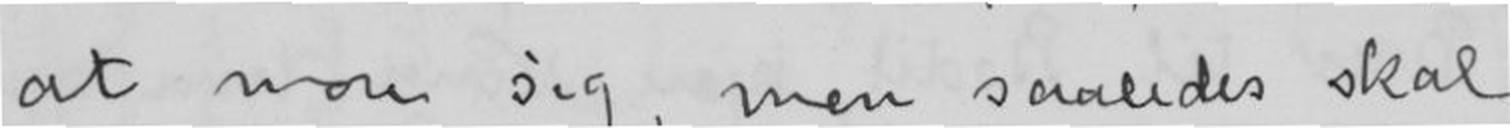at more sig, men saaledes skal
